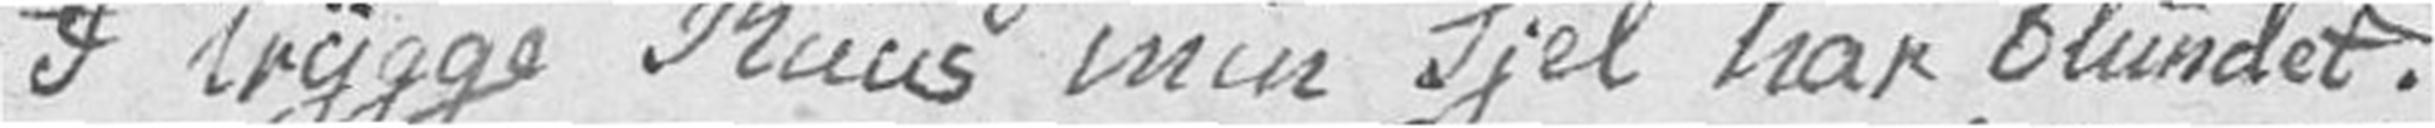I trygge Ruus min Sjel har blundet.
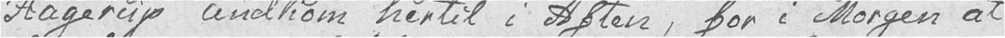Hagerup andkom hertil i Aften, for i Morgen at
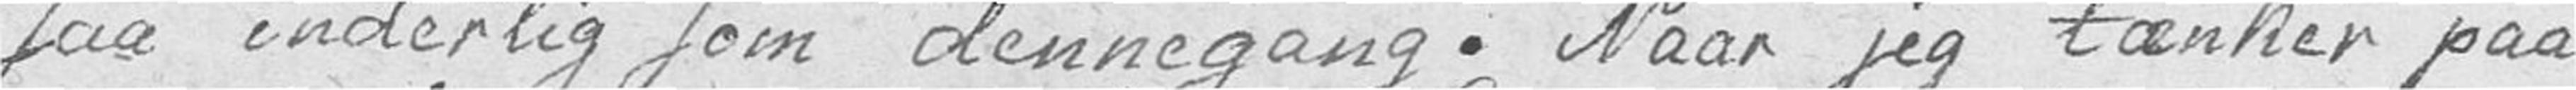saa inderlig som dennegang. Naar jeg tænker paa
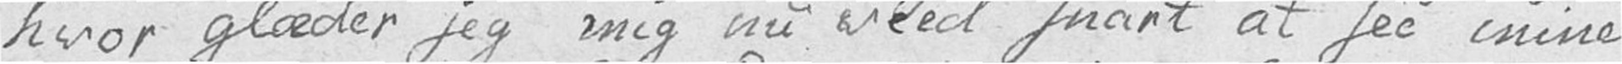hvor glæder jeg mig nu veed snart at see mine
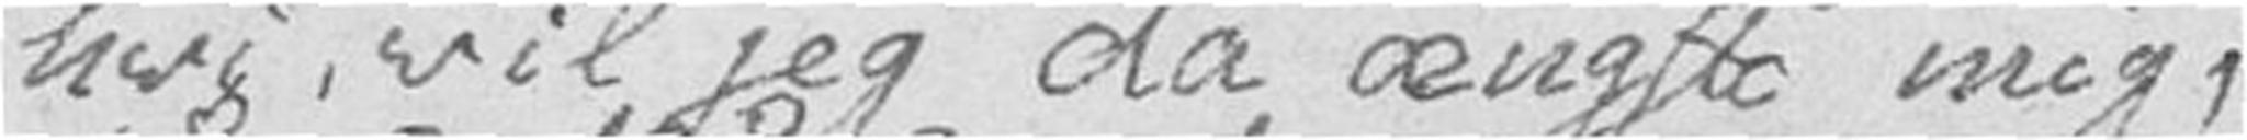hvi, vil jeg da ængste mig,
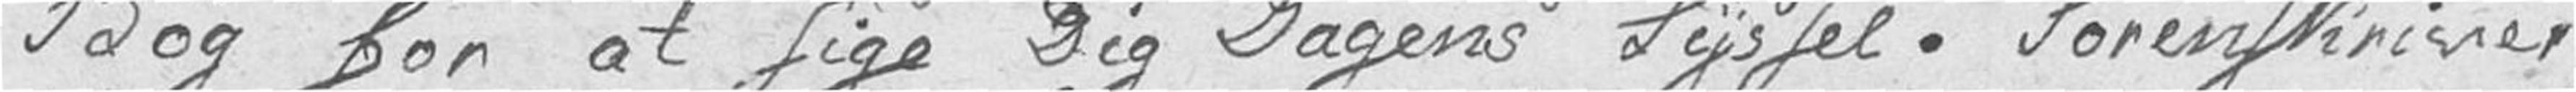Bog for at sige Dig Dagens Syssel. Sorenskriver
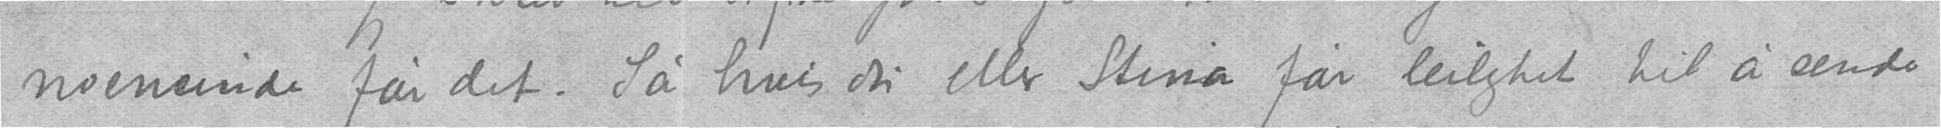noensinde får det. Så hvis du eller Stina får leilighet til å sende
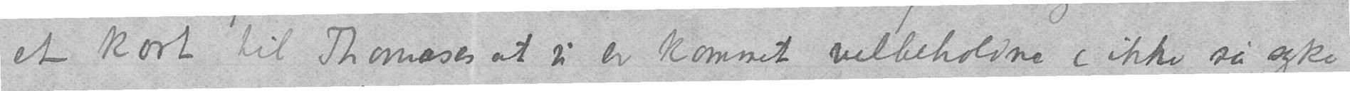et kort til Thomases at vi er kommet velbeholdne (ikke så syke
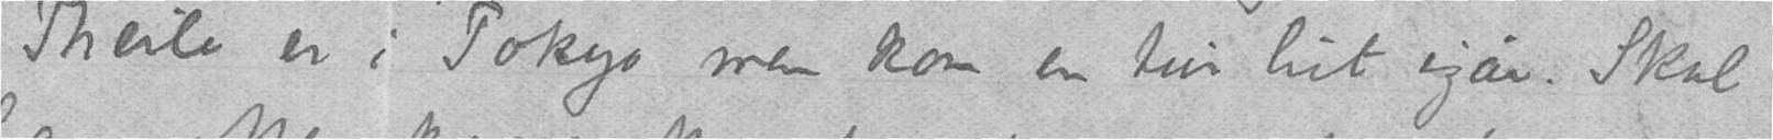Theile er i Tokyo men kom en tur hit igår. Skal
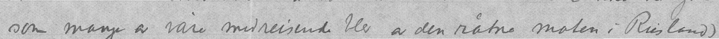som mange av våre medreisende blev av den råtne maten i Rusland)
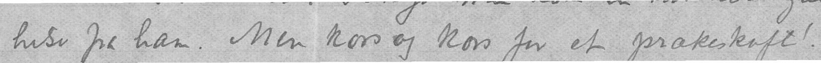hilse fra ham. Men kors og kors for et prækeskaft!.
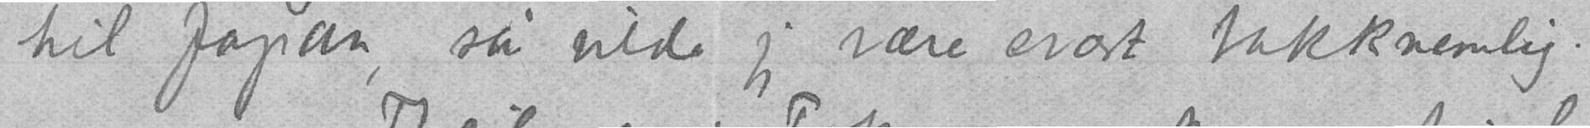til Japan, så vilde jeg være svært takknemlig.
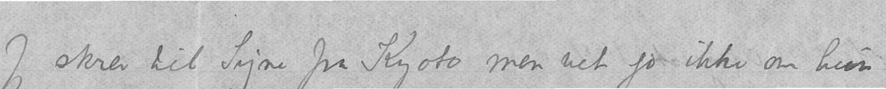Jeg skrev til Signe fra Kyoto men vet jo ikke om hun
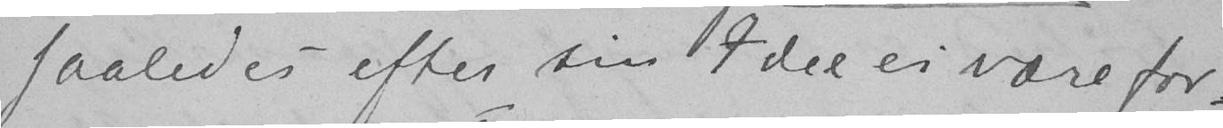saaledes efter sin Idee ei være for-
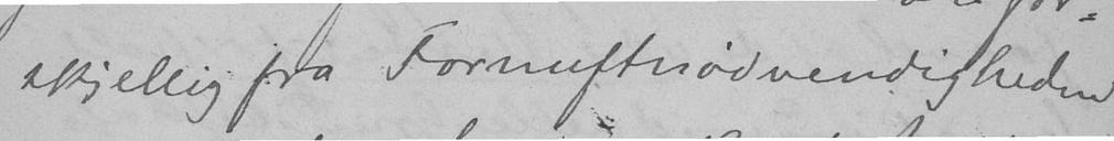skjellig fra Fornuftødvendigheden
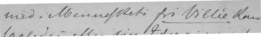med. Menneskets fri villie kan
